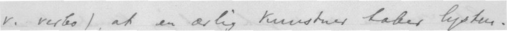v. verbo) at en ærlig kunstner taber lysten.
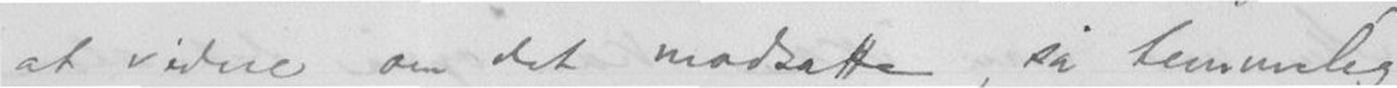at vidne om at modsætte, så temmelig
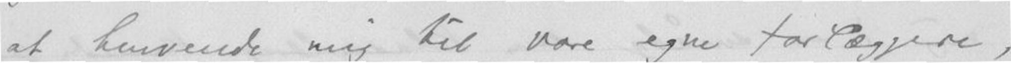at henvende mig til vore egne forlæggere,
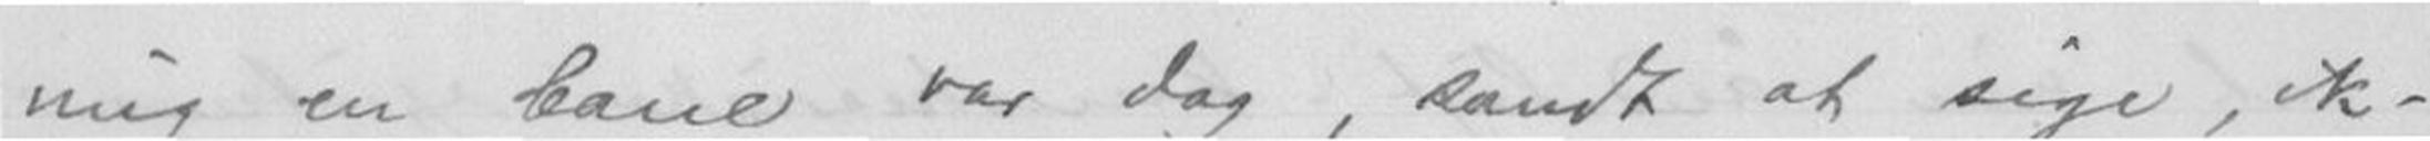mig en bane vor dog, sandt at sige, ik-
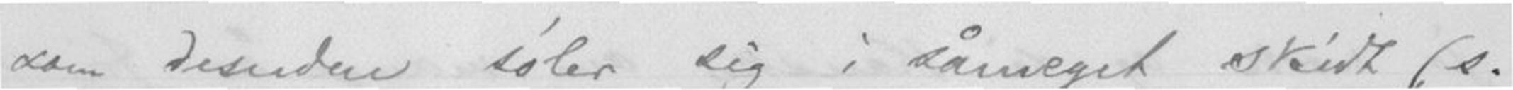som desuden soler sig i såmeget skidt (s.
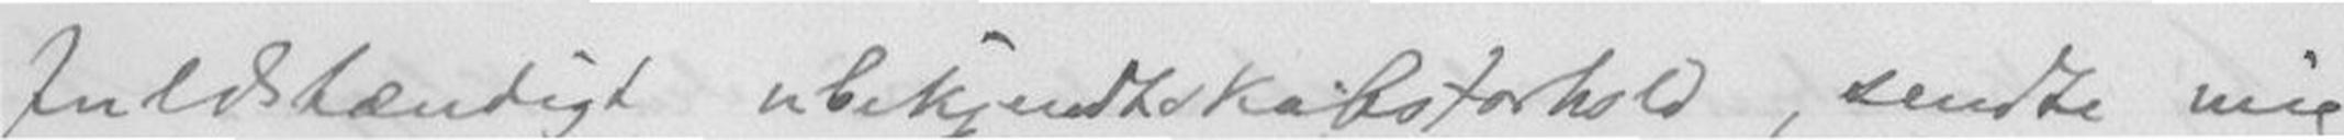fuldstændigt ubekjendtskabsforhold, sendte mig
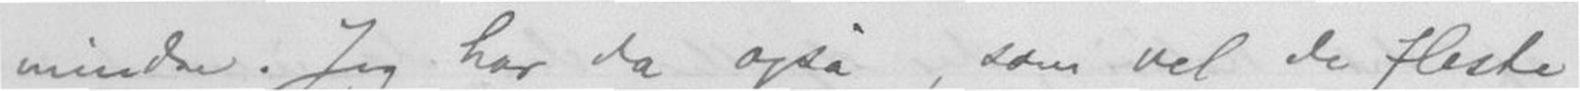mindre. Jeg har da også, som vel de fleste
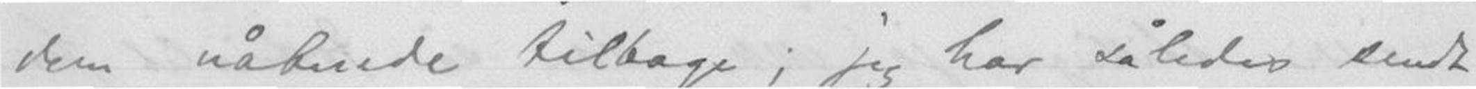dem uåbnede tilbage; jeg har således sendt
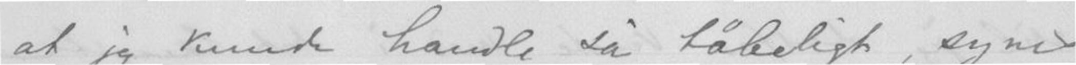at jeg kunde handle så tåbeligt, synes
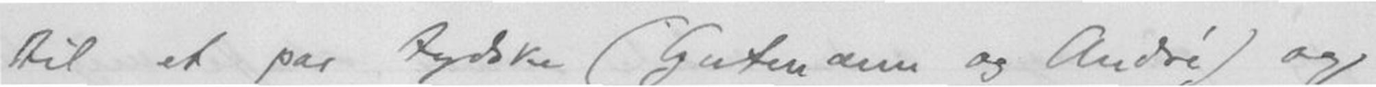til et par tyske (Gutmann og André) og
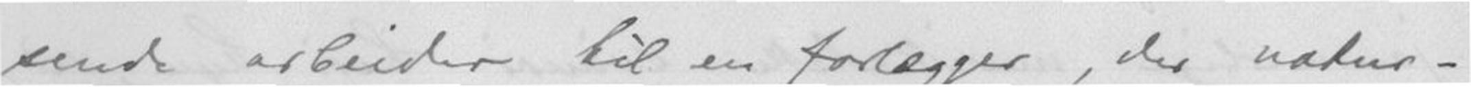sende arbeider til en forlægger, der natur-
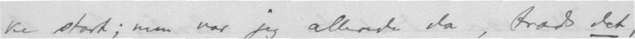ke stort; men vor jeg allerede da, trods det,
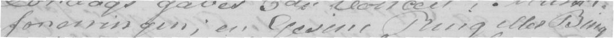foreningen; en Gesine Ring eller Bing
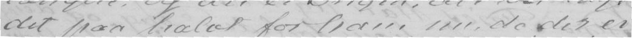det paa halvt for ham nu, da der er
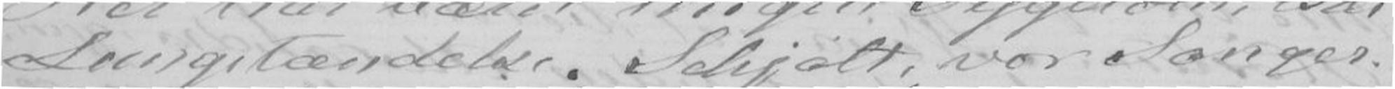Lungebetændelse. Schjøtt, vor Sanger
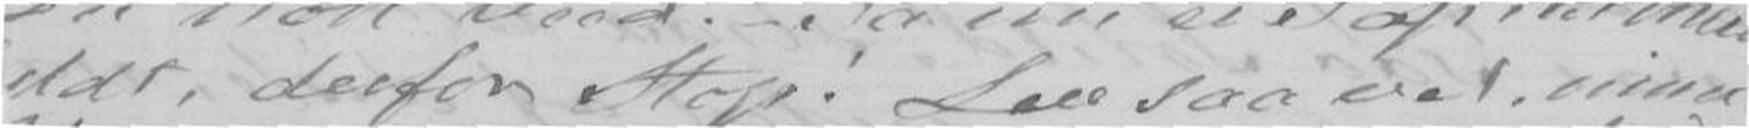eldt, derfor Stop! Lev saa vel, mine
